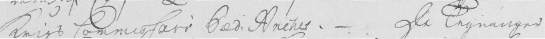Krigs Commissari Ped. Ancher. De Tegninger
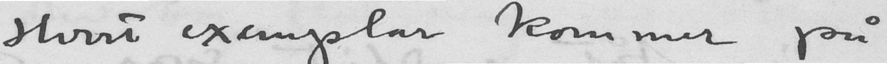Hvert exemplar kommer på
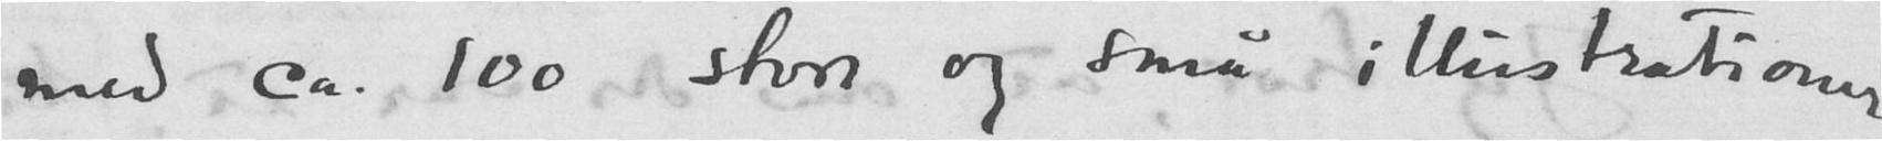med ca. 100 store og små illustrationer
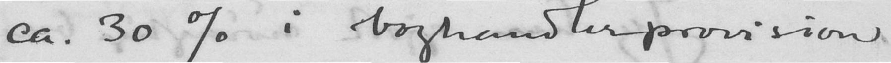ca. 30 % i boghandlerprovision
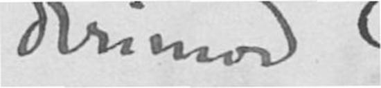derimod
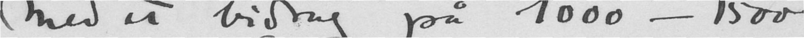Med et bidrag på 1000 - 1500
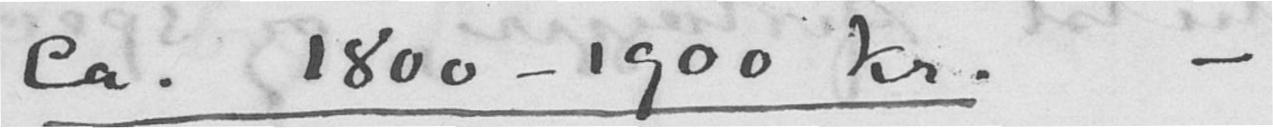ca. 1800-1900 kr. -
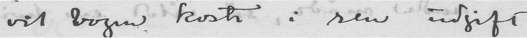vil bogen koste i ren udgift
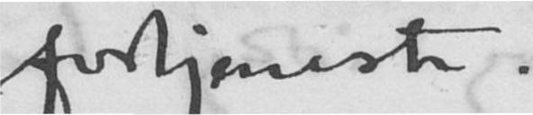fortjeneste.
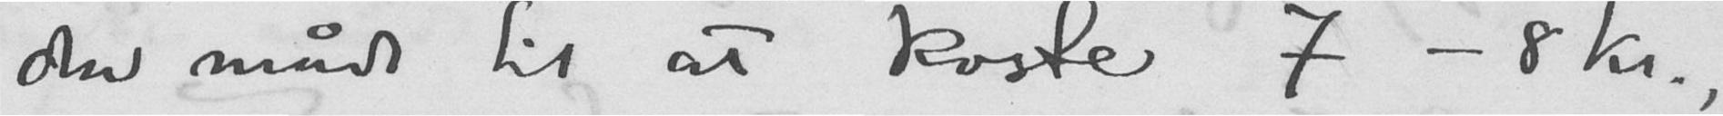den måde til at koste 7 - 8 kr.,
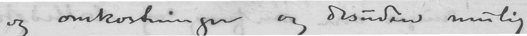og omkostninger og desuden mulig
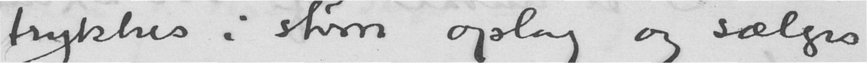trykkes i større oplag og sælges
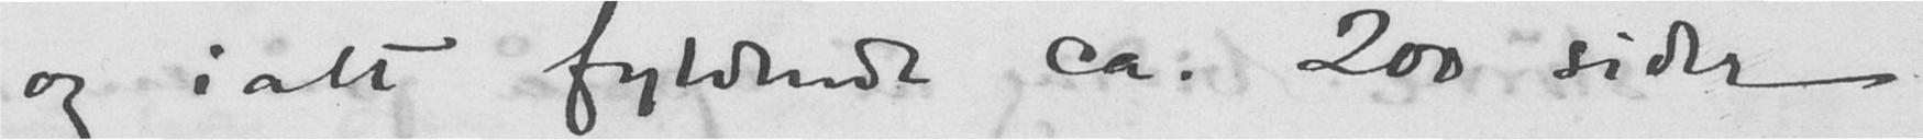og i alt fyldende ca. 200 sider
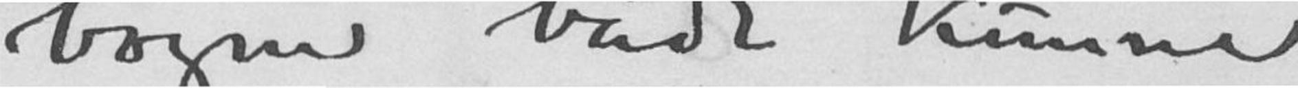bogen både kunne
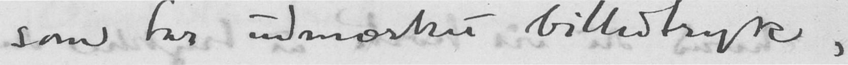som har udmærket billedtryk,
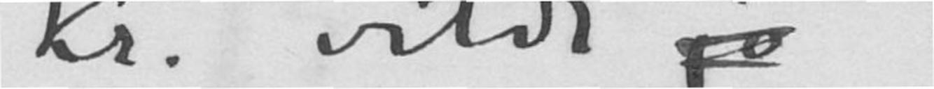kr. vilde jo
